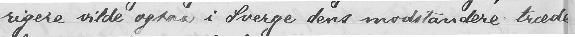rigere vilde ogsaa i Sverge dens modstandere træde
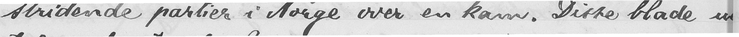stridende partier i Norge over en kam. Disse blade ud-
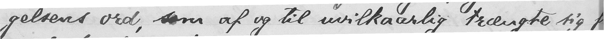gelsens ord, som af og til uvilkaarlig trængte sig
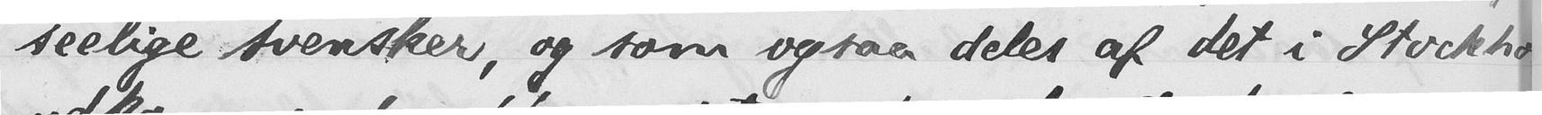seelige svensker, og som ogsaa deles af det i Stockholm
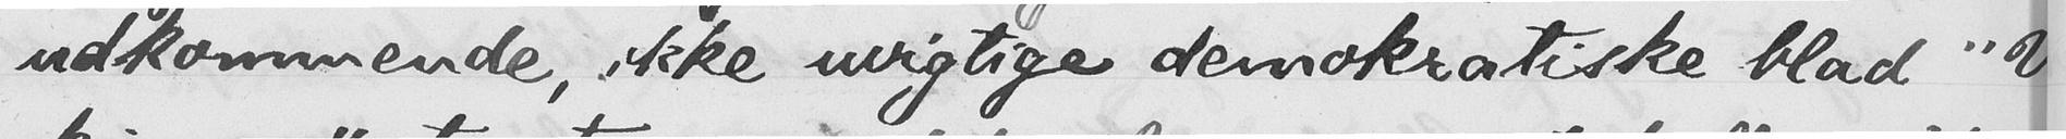udkommende, ikke uvigtige demokratiske blad "
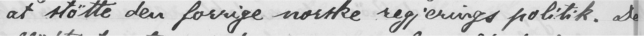at støtte den forrige norske regjerings politik. De
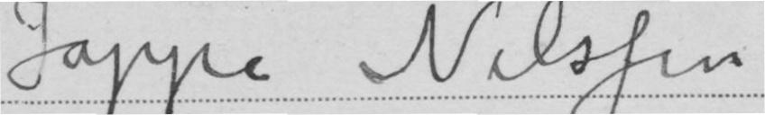Jappe Nilssen
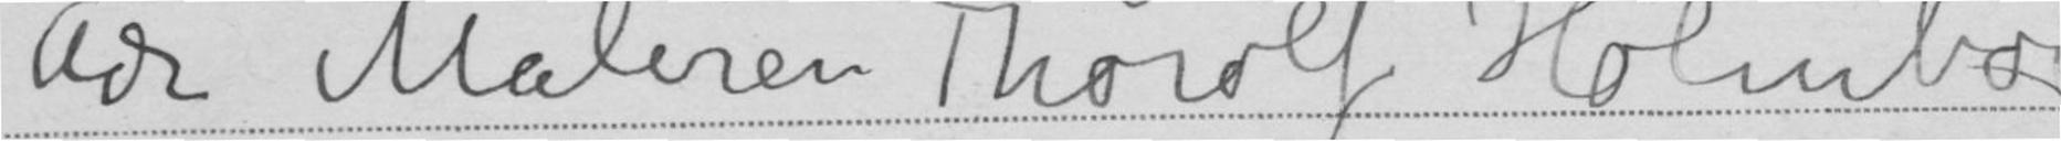Adr. Maleren Thorolf Holmboe
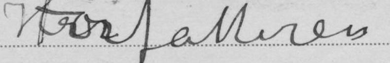Forfatteren
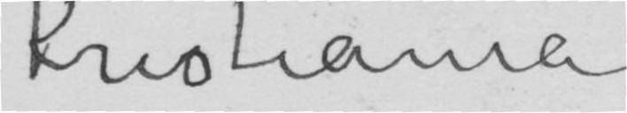Kristiania
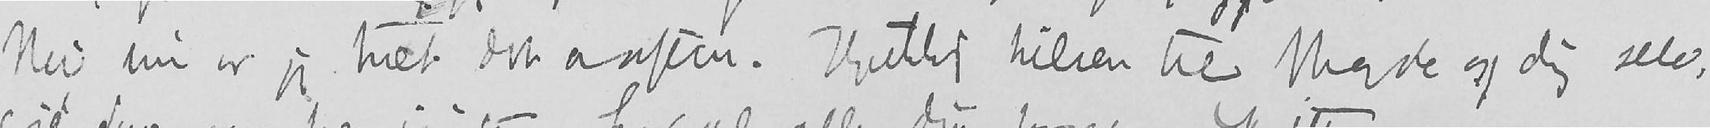Nei nu er jeg træt det er aften. Hjertelig hilsen til Magda og dig selv,
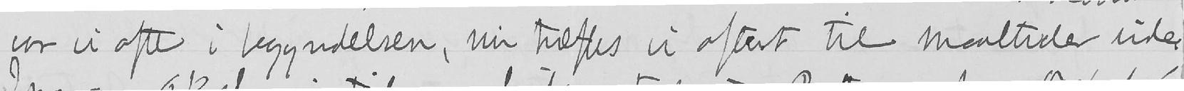var vi ofte i begyndelsen, nu træffes vi oftere til maaltider ude.
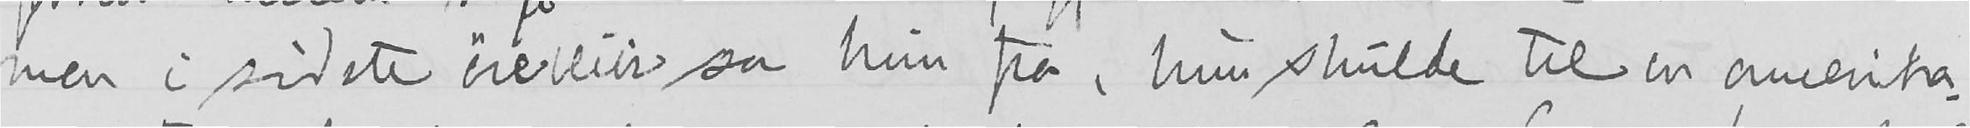men i sidste øieblik sa hun fra, hun skulde til en amerika-
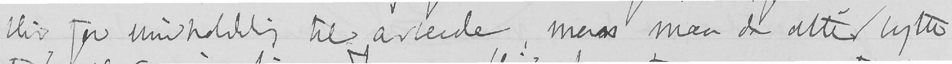blir for uudholdelig til arbeide, man maa da altid bytte
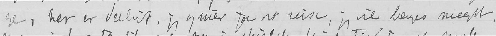ge, her er deiligt, jeg gruer for at reise, jeg vil længes meget,
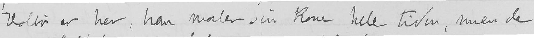Holbø er her, han maler sin kone hele tiden, men de
